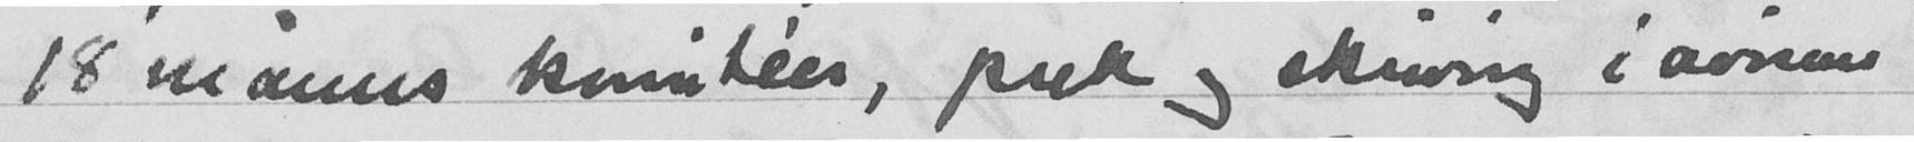18 manns komitèer, prek og skriving i avisene
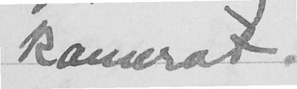kamerat.
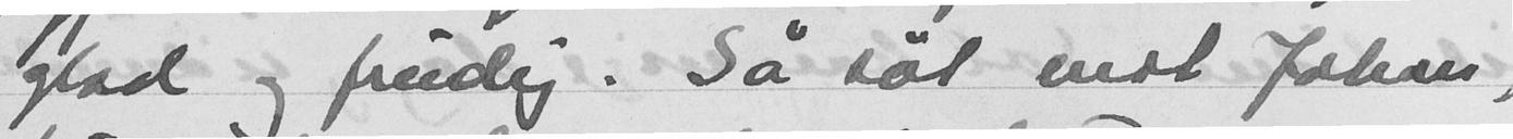glad og freidig. Så søt mot Johan,
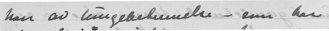han av lungebetennelse - som har
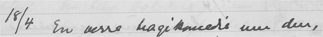18/4 En verre tragikomedie enn den,
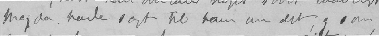Magda havde sagt til ham om det, og som
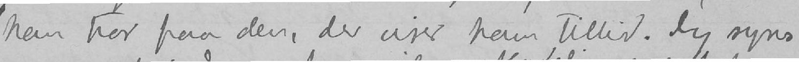han tror paa den, der viser ham tillid. Jeg synes
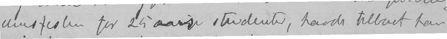umsfesten for 25 aarige studenter, havde tilbudt han
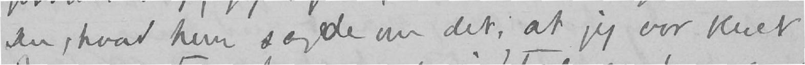Du, hvad hun sagde om det, at jeg var blivet
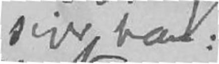siger han:
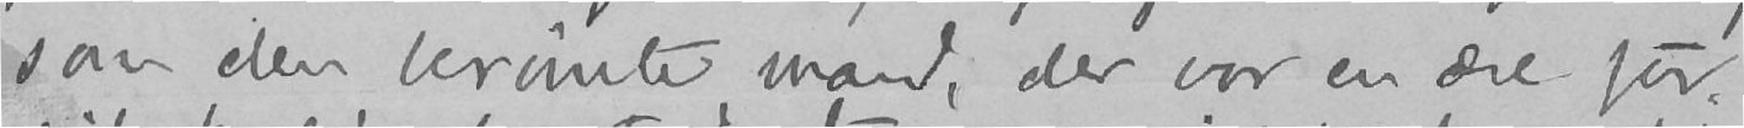som den berømte mand, der var en ære for
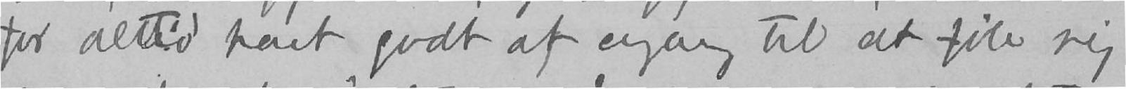for alltid havt godt af engang til at føle sig
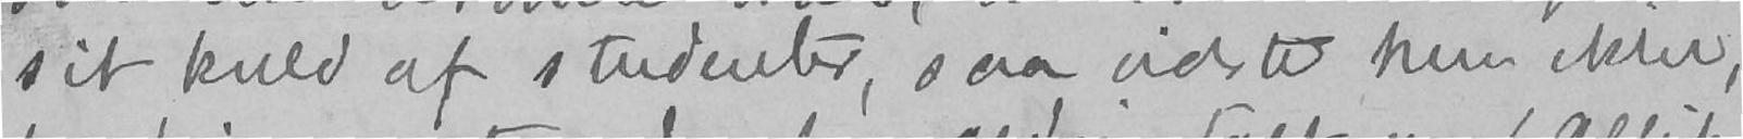sit kuld af studenter, saa vidste hun ikke,
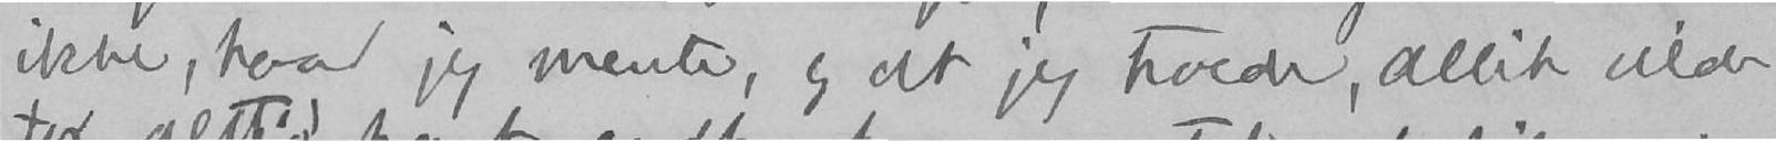ikke, hvad jeg mente, og at jeg troede, Allik vilde
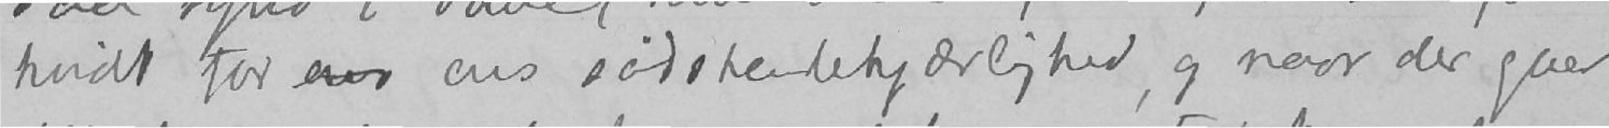hvidt ans ens sødskendekjærlighed, og naar der gaaer
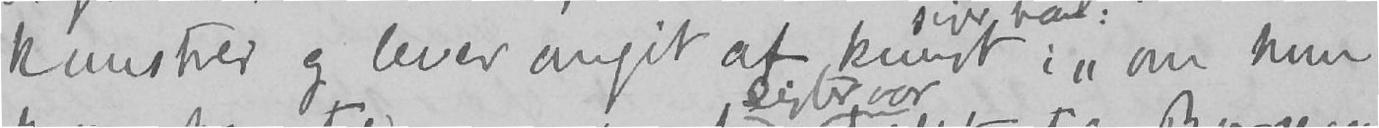kunstner og lever omgit af kunst; «om hun
